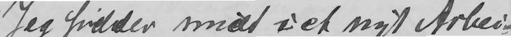Jeg sidder mid i et nyt Arbei-
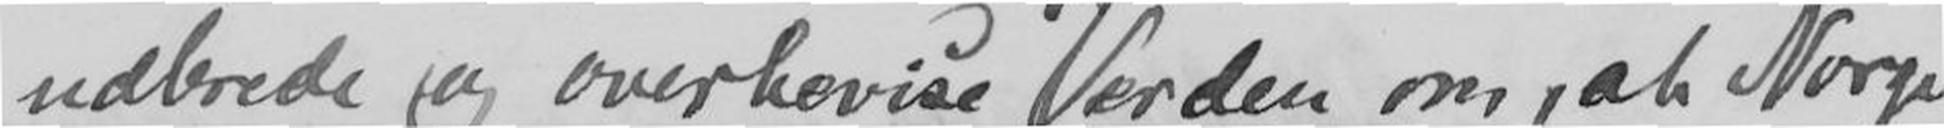udbrede og overbevise Verden om, at Norge
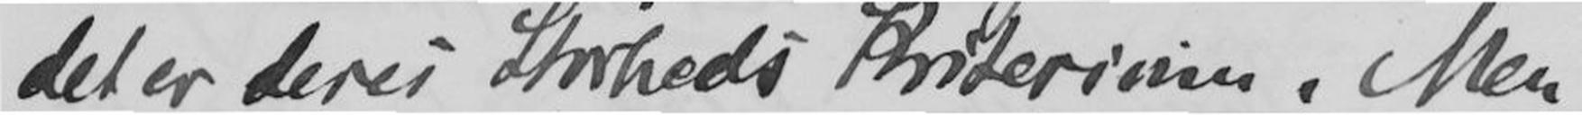det er deres Storheds Kriterium. Men
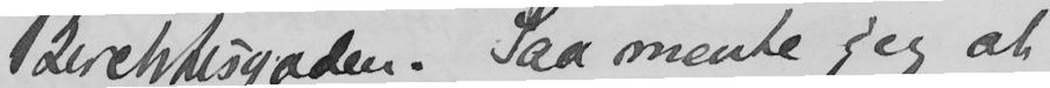Birekkesgaden. Saa mente jeg at
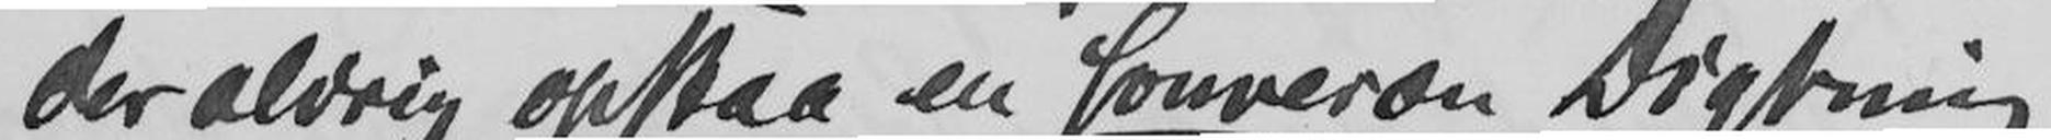der aldrig opstaa en Souveræn Digtning
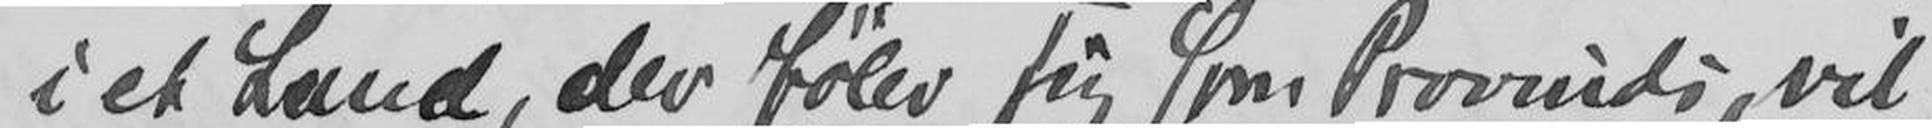i et Land, der føler sig som Provinds, vil
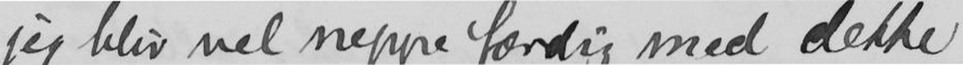og jeg blir neppe færdig med dette
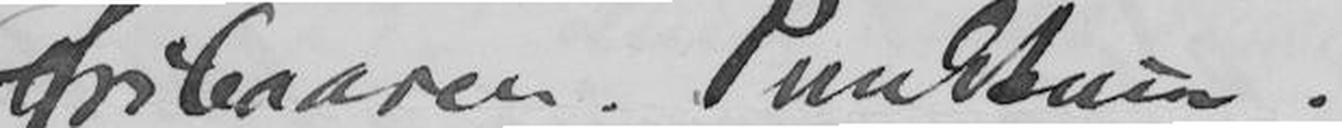fribaaren. Punktum.
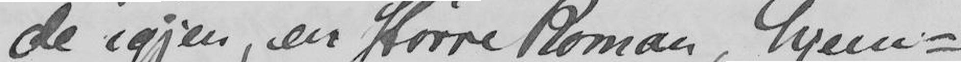de igjen, en større Roman, hjem-
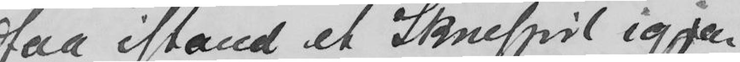faa istand et Skuespil igjen,
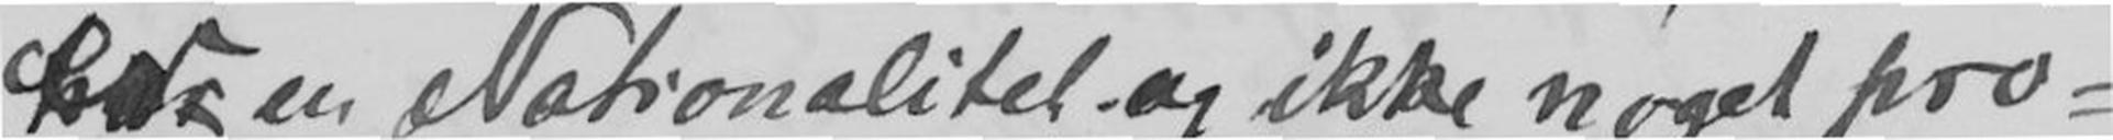har en Nationalitet og ikke noget pro-
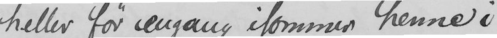heller før engang isommer henne i
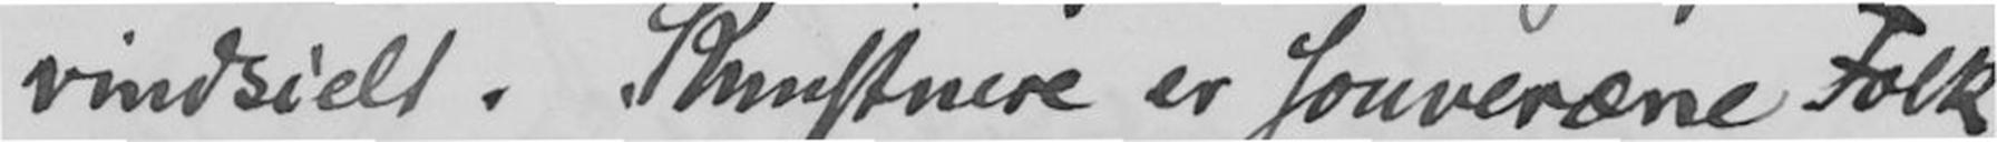vindsielt. Kunstnere er souveræne Folk,
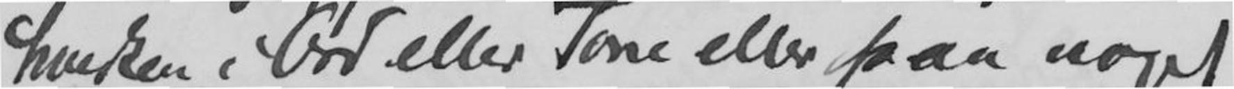hverken i Ord eller Tone eller paa noget
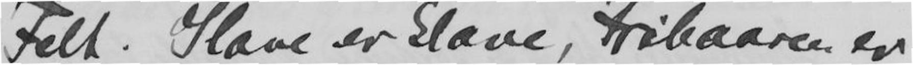Felt. Slave er Slave, Fribaaren er
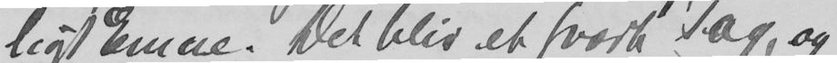ligt Emne Det blir et svært Tag,
